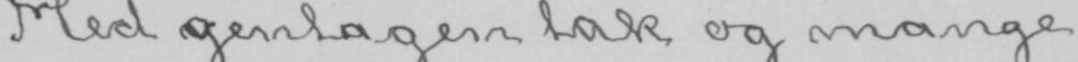Med gentagen tak og mange
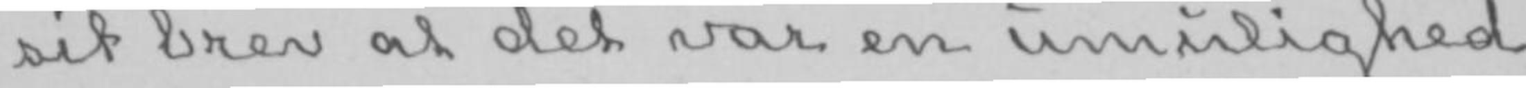sit brev at det var en umulighed
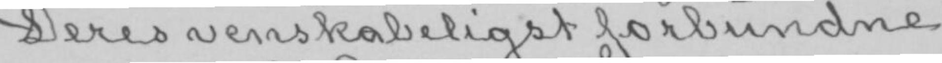Deres venskabeligst forbundne
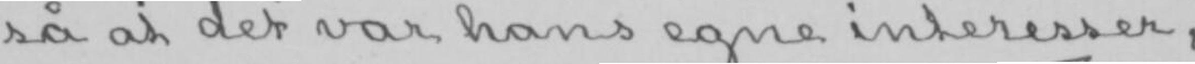så at det var hans egne interesser,
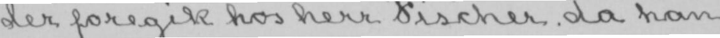der foregik hos herr Fischer da han
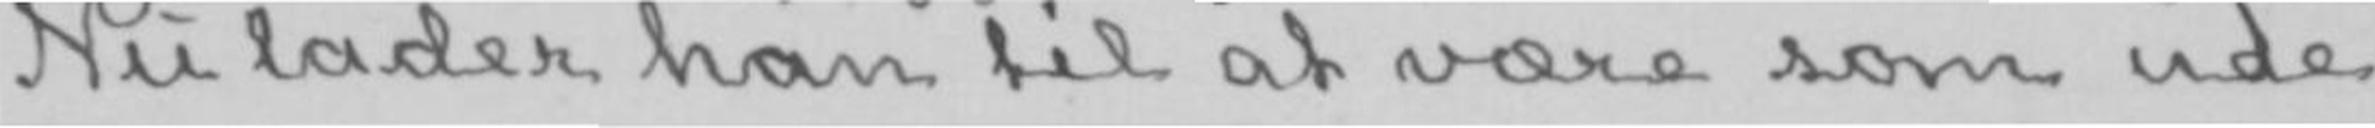Nu lader han til at være som ude
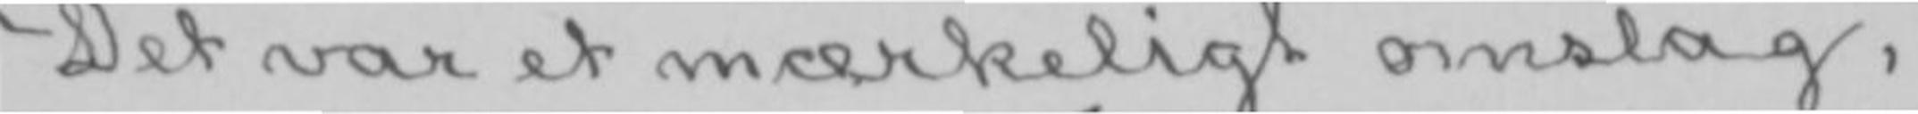Det var et mærkeligt omslag,
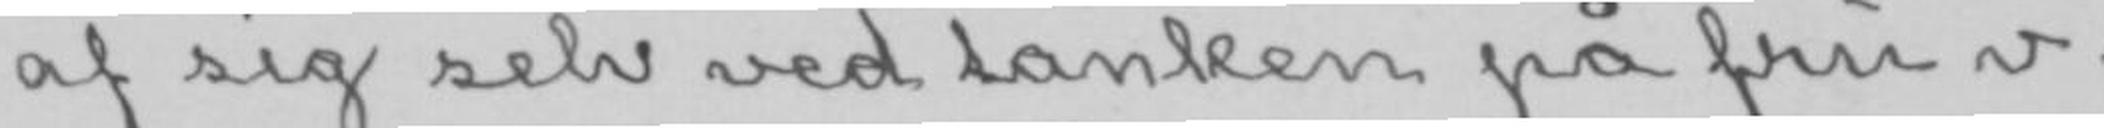af sig selv ved tanken på fru v.
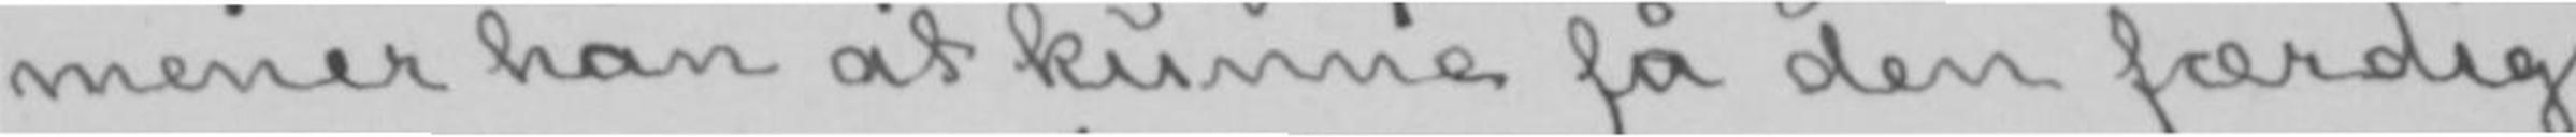mener han at kunne få den færdig
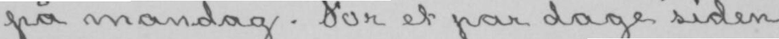på mandag. For et par dage siden
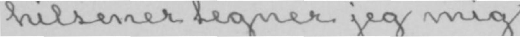hilsener tegner jeg mig
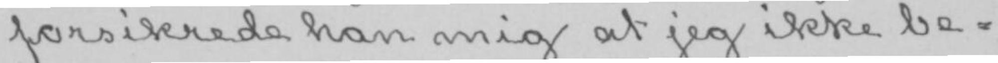forsikrede han mig at jeg ikke be-
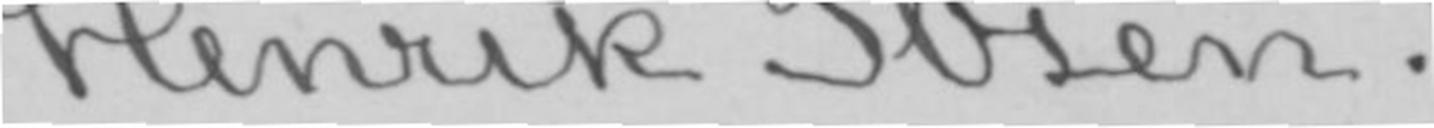Henrik Ibsen.
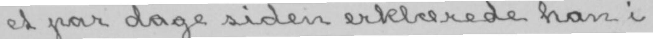et par dage siden erklærede han i
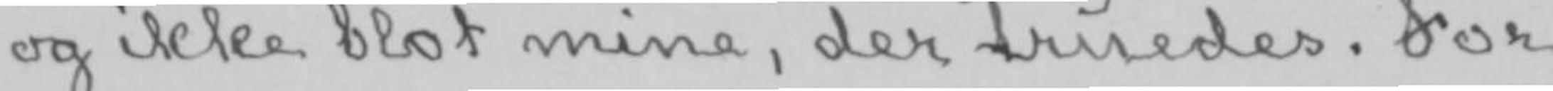og ikke blot mine, der truedes. For
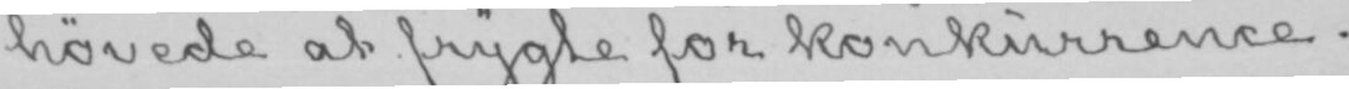høvede at frygte for konkurrence.
